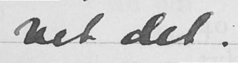vet det.
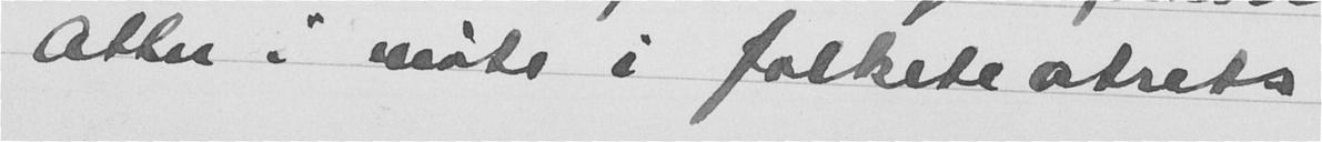atter i møte i folketeatrets
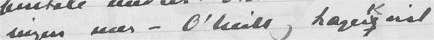ingen mer - O'keith og Lagerkvist
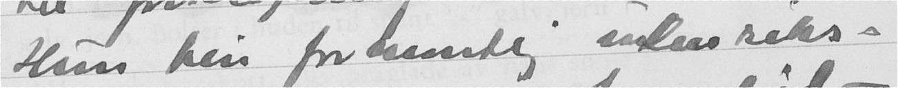Hun blir formentlig utenriks-
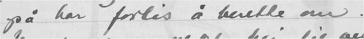også har forlis å berette om.
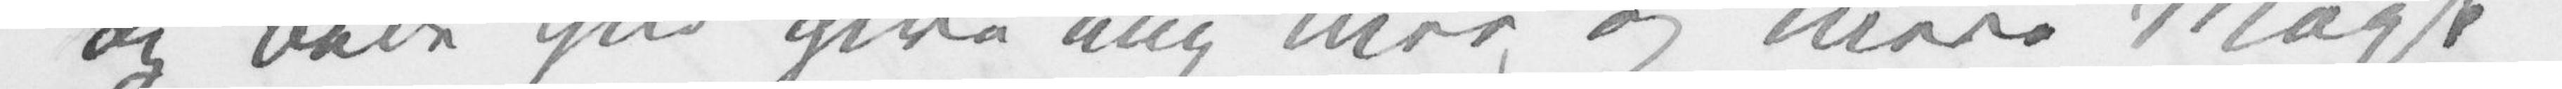og bede Gud give mig mer og mere Magt
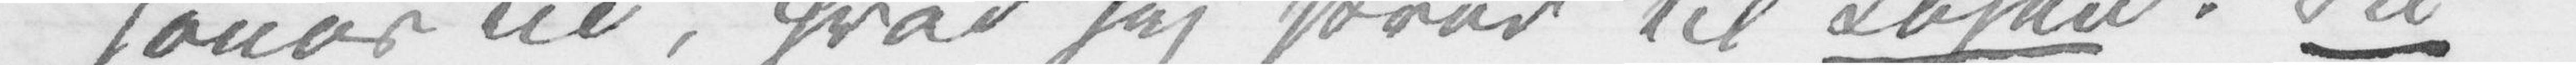Jonas Lie, hvad jeg skrev til Ibsen. Så
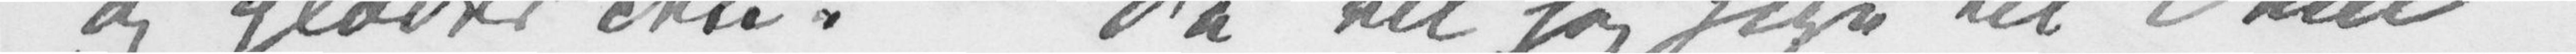og glæder den! Så vil jeg sige til Dem
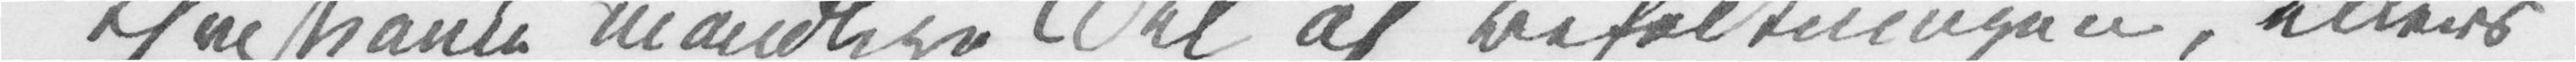Christiania[s] mandlige Del af Befolkningen, ellers
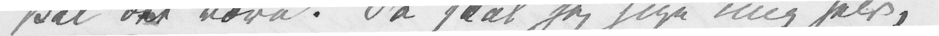skal det være ! Så skal jeg sige mig selv,
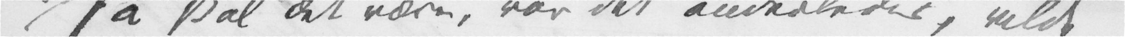så skal det være, var det anderledes, vilde
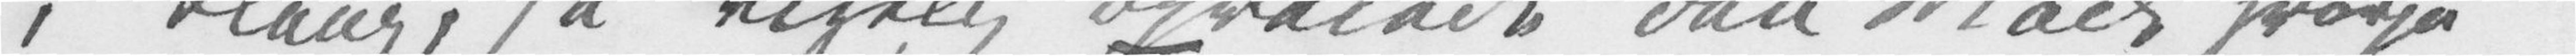i Klang, så rigelig opveiede den Måde hvorpå
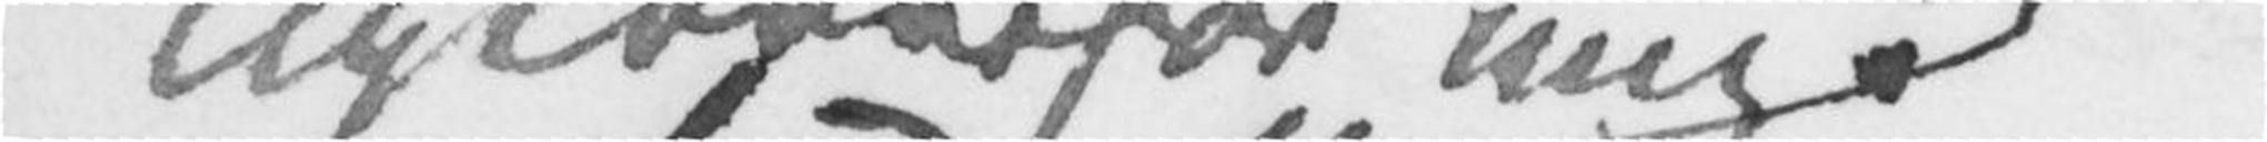ligeoverfor mig:
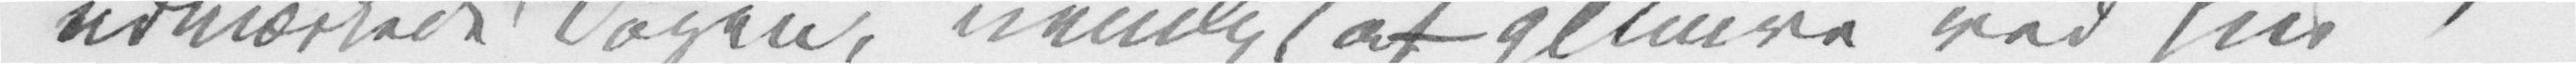udmærkede Dagen, nemlig at glimre ved sin
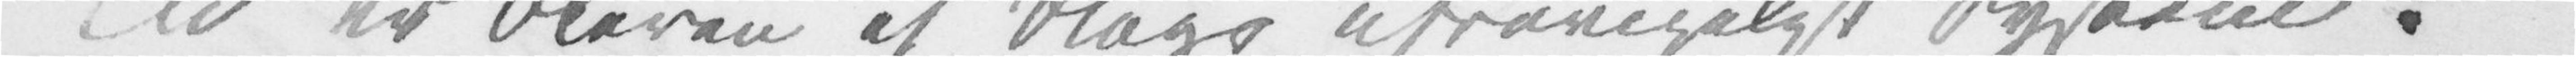Tid er bleven et Slags ufravigeligt System:
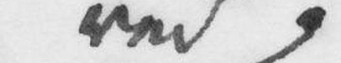ved
